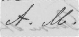A. M.
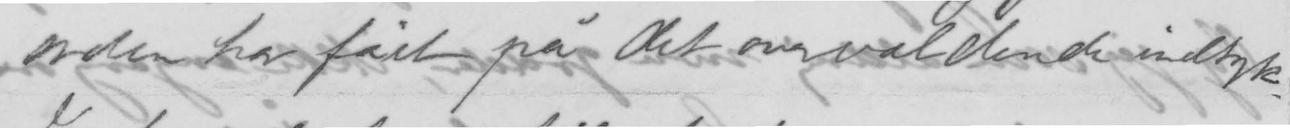orden har fået på det overvældende indtryk.
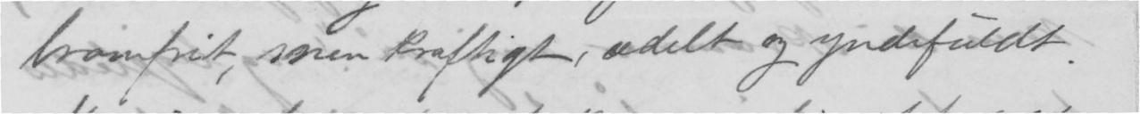bramfrit, men kraftigt, ædelt og yndefuldt.
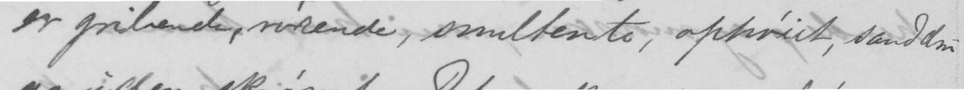er gribende, rørende, smeltente, ophøiet, sanddru
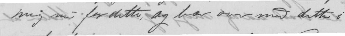mig nu for dette, og bær over med dette i
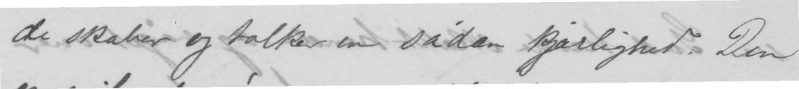de skaber og tolker en sådan kjærlighet. Den
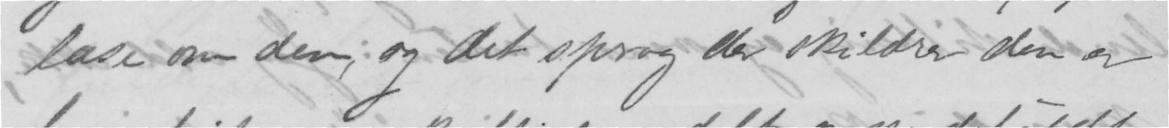læse om den, og det sprog der skildrer den er
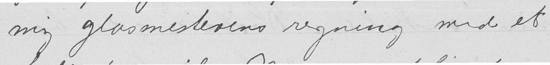mig glasmesterens regning med et
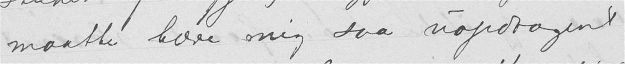maatte bære mig saa uopdragent
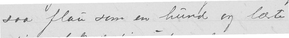saa flau som en hund og læste
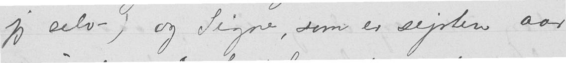jeg selv) og Signe, som er sejsten aar
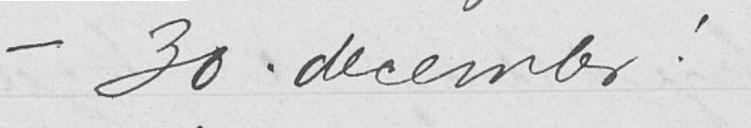- 30.december!
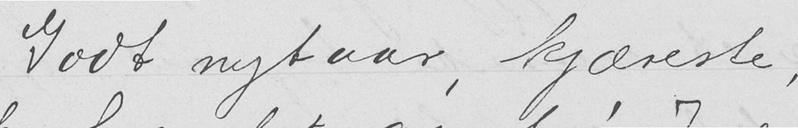Godt nytaar, kjæreste,
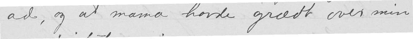ad, og at mama havde grædt over min
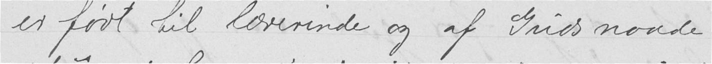er født til lærerinde og af Guds naade
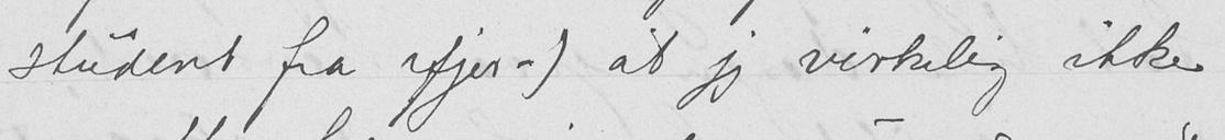student fra ifjor -) at jeg virkelig ikke
